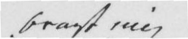bragt mig
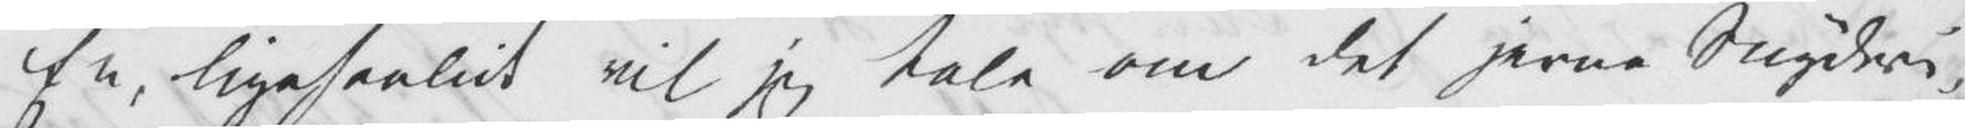En, ligesaalidt vil jeg tale om det jevne Snyderi
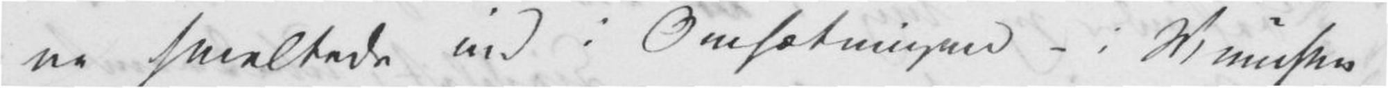ne smeltede ind i Omsætningen – i München
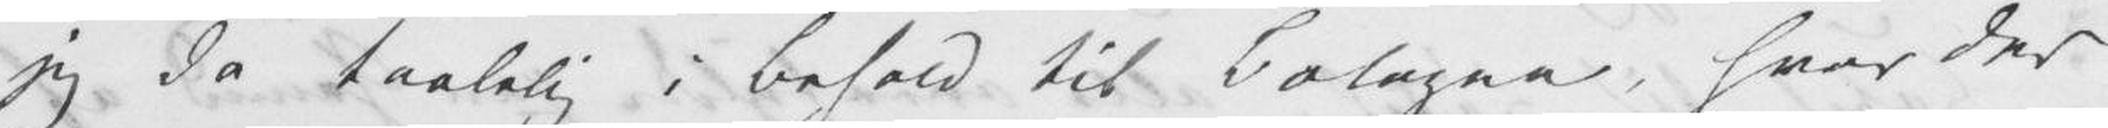jeg da taalelig i Behold til Bologna, hvor der
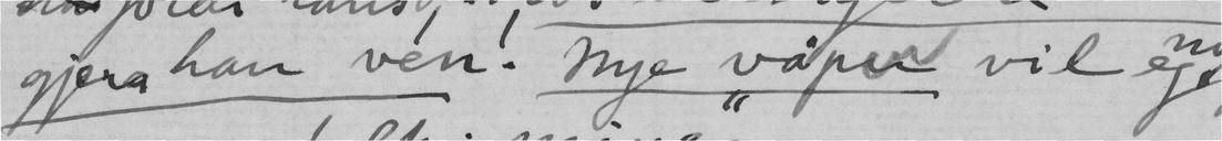gjera han ven! nye våpen vil eg
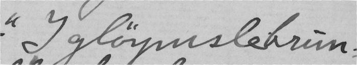"I gløymslebrun-
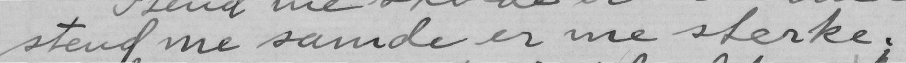stend me samde er me sterke;
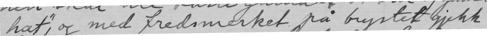hat", og med fredsmerket på brystet gjekk
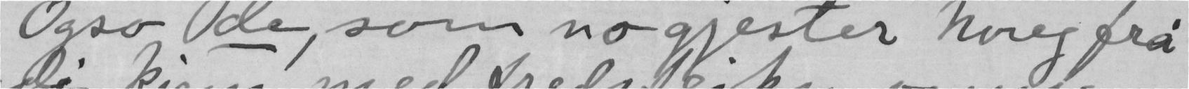Ogso de, som no gjester Noreg frå
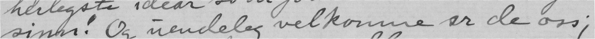sinn! Og uendeleg velkomne er de oss;
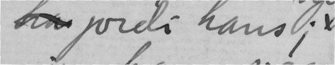fra jordi hans;
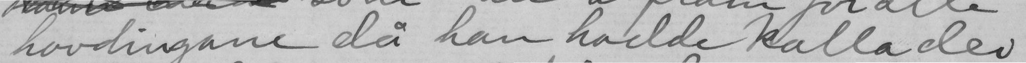hovdingane då han hadde kalla dei
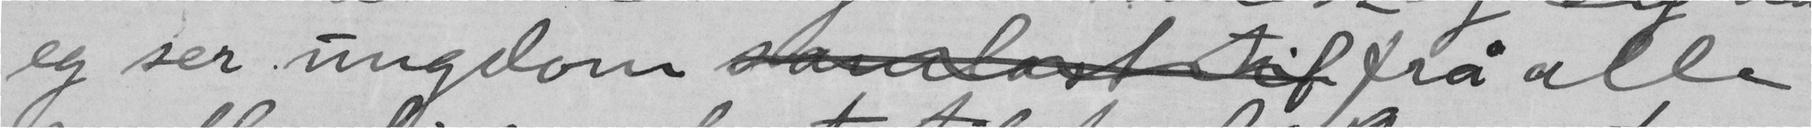eg ser ungdom samlast til frå alle
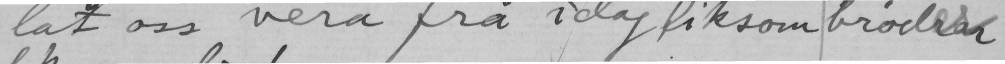lat oss vera fra i dag liksom brødrar
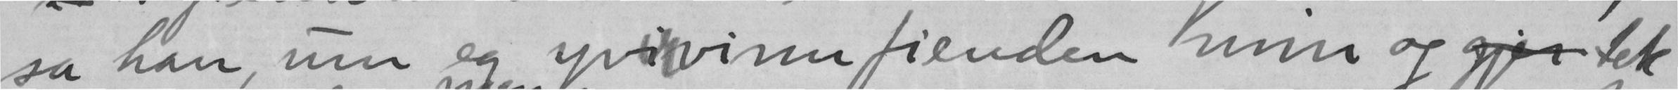sa han, um eg yvivinn fienden min og gjer tek
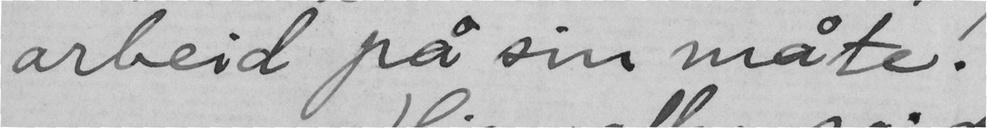arbeid på sin måte.
